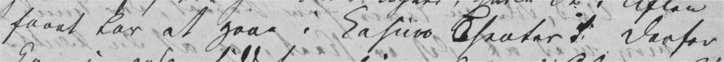faaet Lov at gaae i Kasino Theater! Derfor
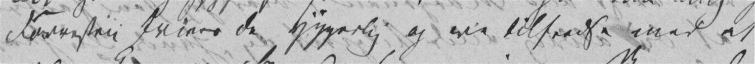Forresten trives de ypperlig og ere tilfredse med at
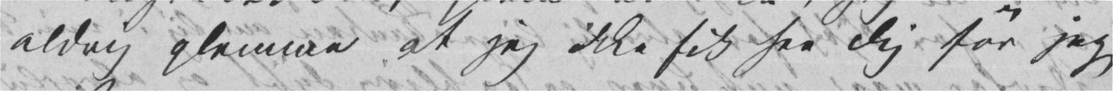aldrig glemme at jeg ikke fik see Dig før jeg
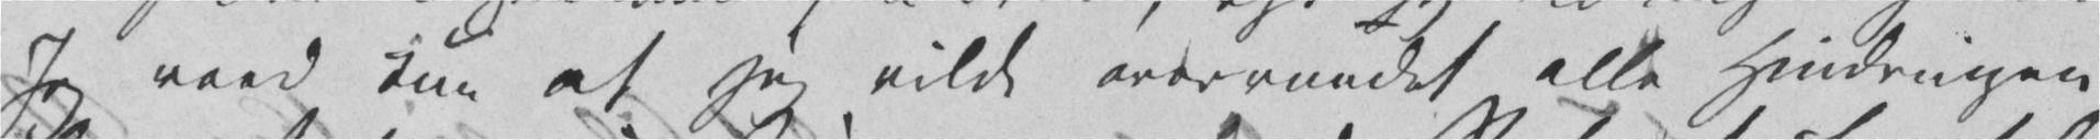Jeg veed kun at jeg vilde overvundet alle Hindringer
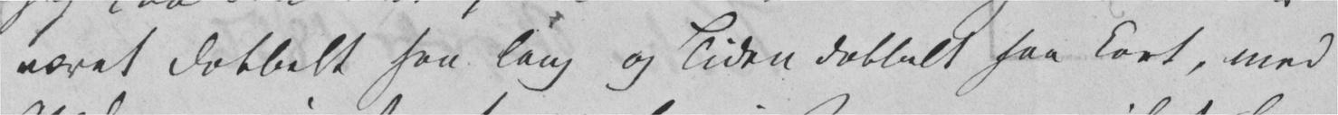været dobbelt saa lang og Tiden dobbelt saa kort, med
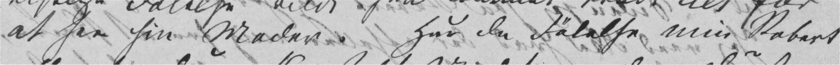at see sin Moder. Har Du Følelse min Robert
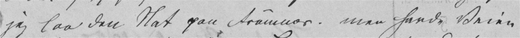jeg laa den Nat paa Framnæs. men havde Veien
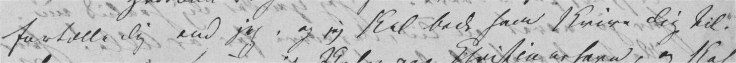fortælle Dig end jeg, og jeg skal bede ham skrive Dig til.
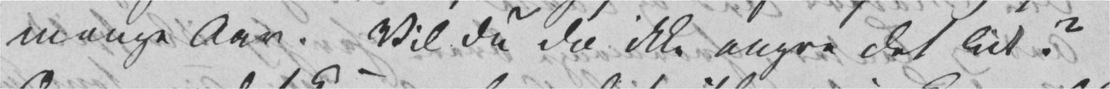mange Aar. Vil Du da ikke angre det lidt?
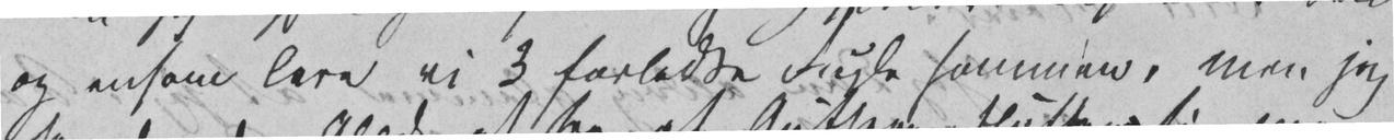og ensom leve vi 3 forladte Fugle sammen, men jeg
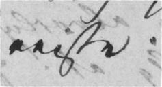reiste.
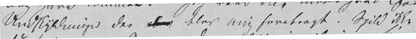Undskyldninger der <gap/> blev mig forebragt. Spild ikke
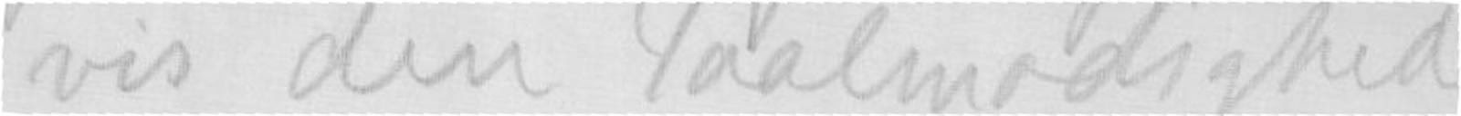vis den Taalmodighed
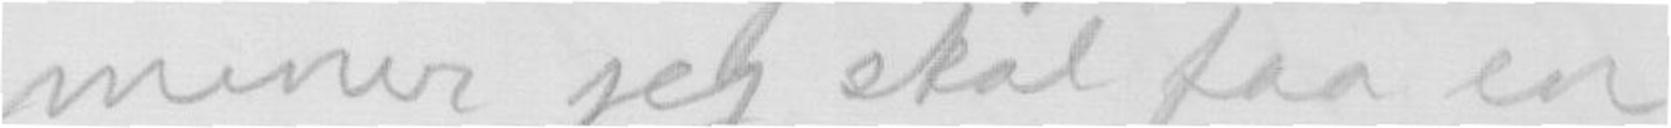mener jeg skal faa en
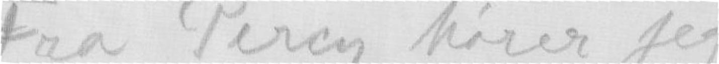Fra Percy hører jeg
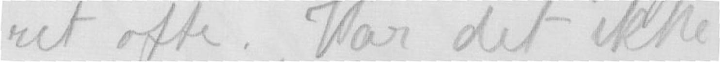ret ofte. Var det ikke
